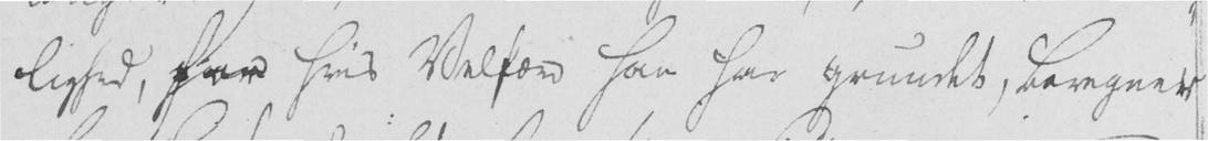lighed, for hvis Velfærd han har grundet, beregnede
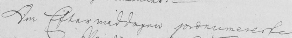Om Eftermiddagen prænumererte
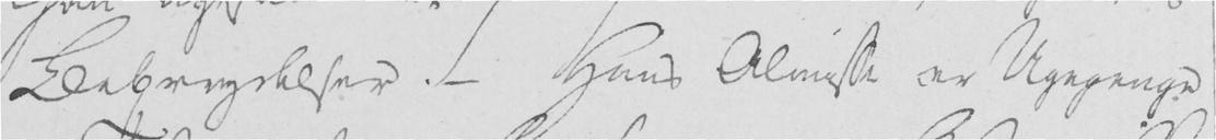Bebreydelser. - Hans Almisse er Ugegenge
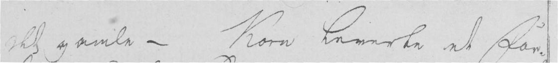det gamle - Korn leverte et For-
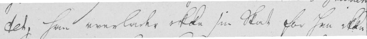tet, han overlader ikke sin Skat for han ikke
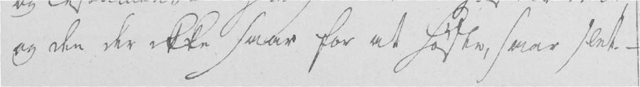og den der ikke saar for at høste, saar slet. -
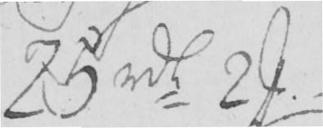25 rde 2 s. -
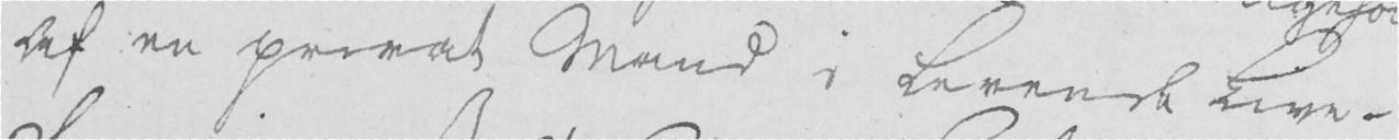af en privat Mand i levende live -
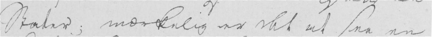Stater; mærkelig er det at see en
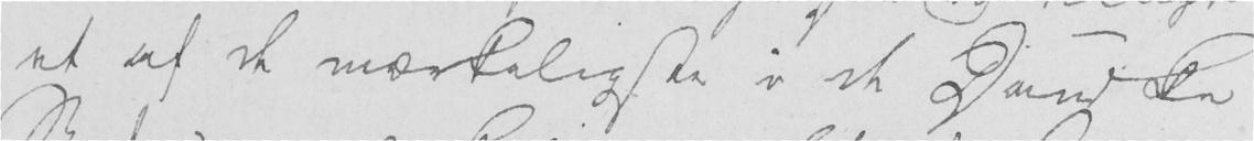et af de mærkeligste i de Danske
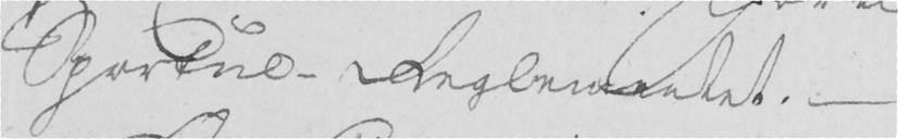Sportul-Reglementet. -
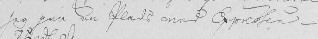jeg paa en Plads med Expressen -
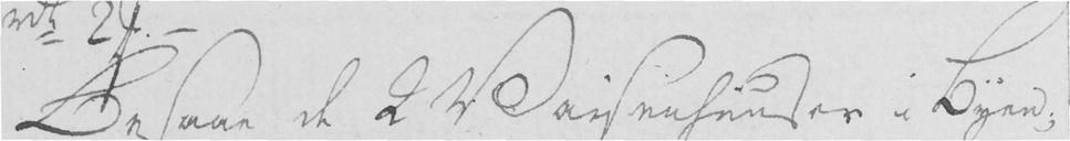Besaae de 2 Vaisenhuuser i Byen;
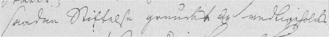saadan Stiftelse grundes og vedligeholdes
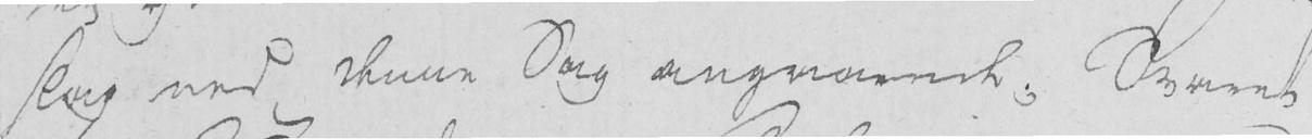slag ved denne Sag angaaende; Svaret
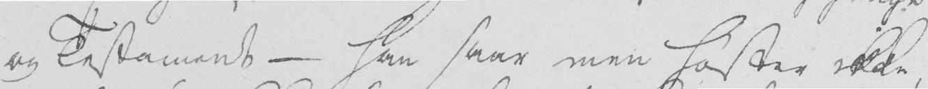og Testament - han saar men høster ikke,
